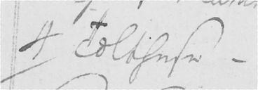4 Tælthuse -
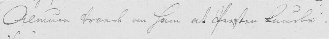Almuen troede om ham at Præsten kunde
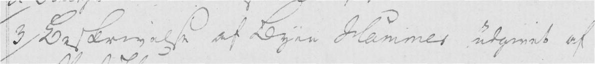3 Beskrivelse af Byen Hammer udgivet af
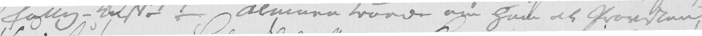fally-Erse - Almuen troede om ham at Provsten
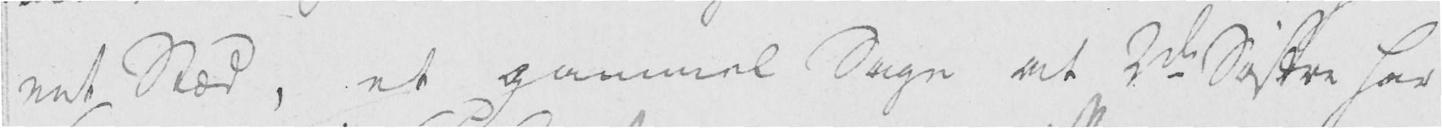eet Stæd, et gammel Sagn at 2de Søstre har
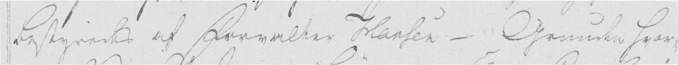bestyredes af Forvalter Hansen - Grunden hvor-
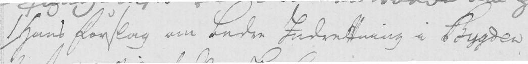1 Hans forslag om bedre Indrettning i Bygden
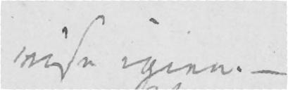vise igien.
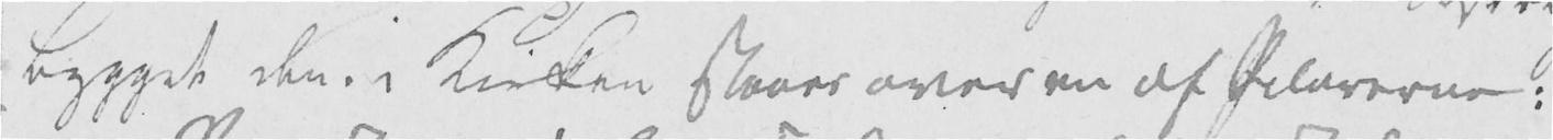bygget den. i Kirken staaer over en af Pilarerne:
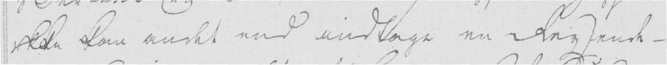ikke kan andet end indtage en Reysende -
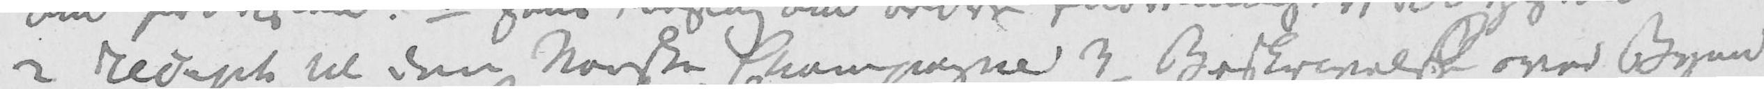2 recept til den Norske Champagne 3 Beskrivelse over Byen
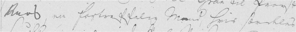Aars en fortreffelig Mand, hvis særdeles
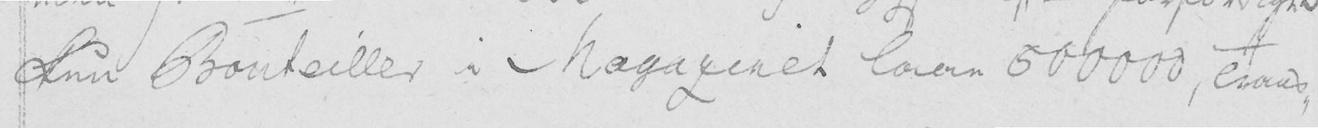kun Bouteiller i Magazinet laae 500000, Trans-
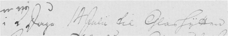i 2 Dage 14 Julii til Glashytten
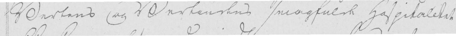Vertens og Vertindens smagfulde Hospitalitet
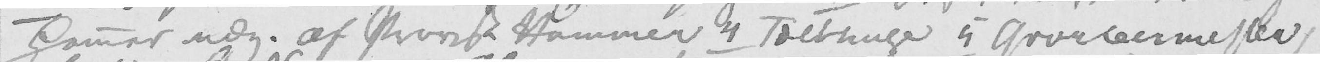Hamar udg. af Provst Hammer 4 Tælthuse 5 Gruvelenmester,
